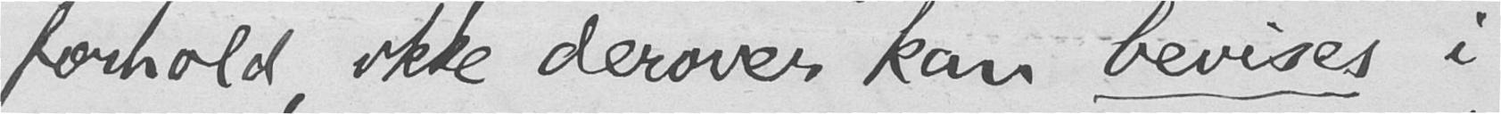forhold, ikke derover kan bevises i
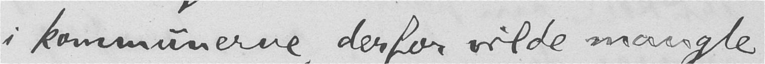i kommunerne, derfor vilde mangle
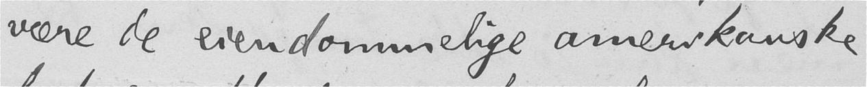være de eiendommelige amerikanske
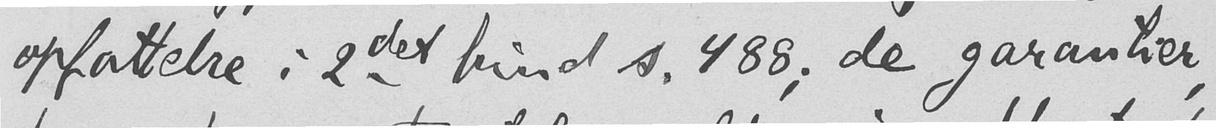opfattelse i 2det bind s. 488; de garantier,
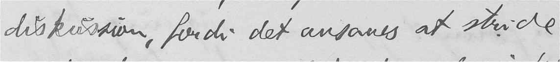diskussion, fordi det ansaaes at stride
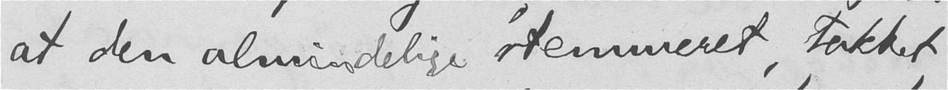at den almindelige stemmeret, takket
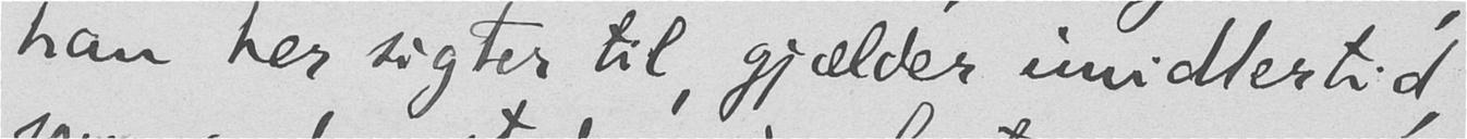han her sigter til, gjælder imidlertid,
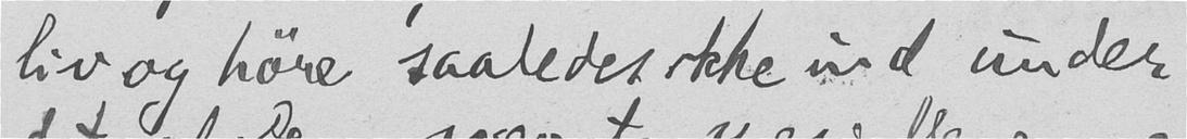liv og høre saaledes ikke ind under
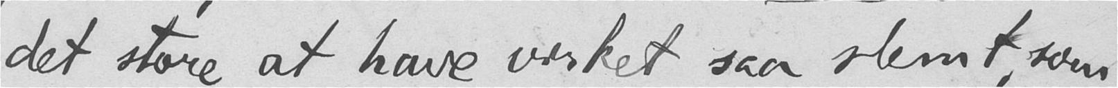det store at have virket saa slemt, som
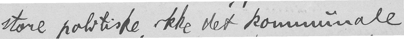store politiske, ikke det kommunale
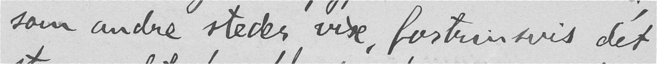som andre steder vise, fortrinsvis det
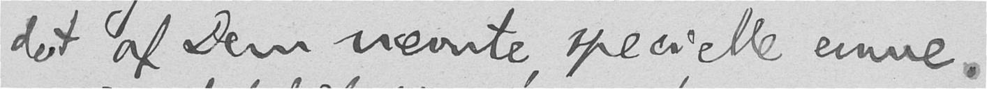det af Dem nævnte, specielle emne.
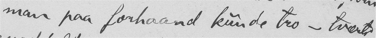man paa forhaand kunde tro – tværti-
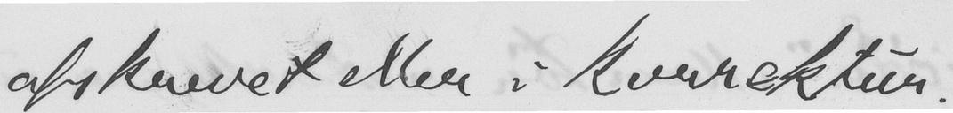afskrevet eller i korrektur.
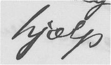hjælp
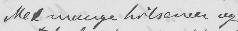Med mange hilsener og
